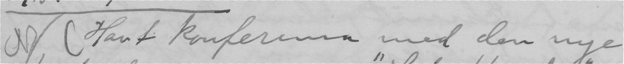Havt konferanse med den nye
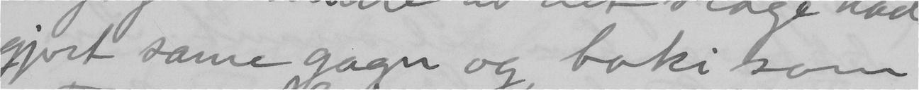gjort same gagn og boki som
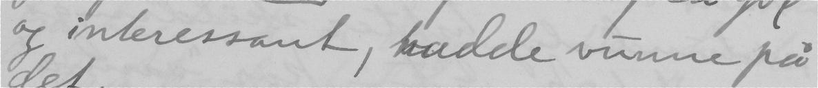og interessant, hadde vunne på
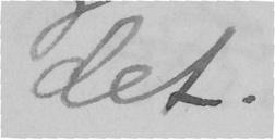det.
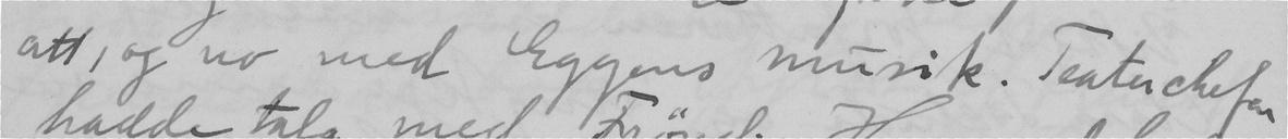att, og no med Eggens musik. Teaterchefen
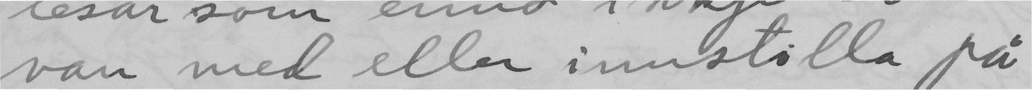van med eller innstilla på
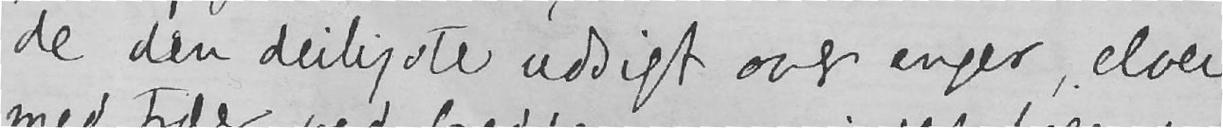de den deiligste udsigt over enger, elver
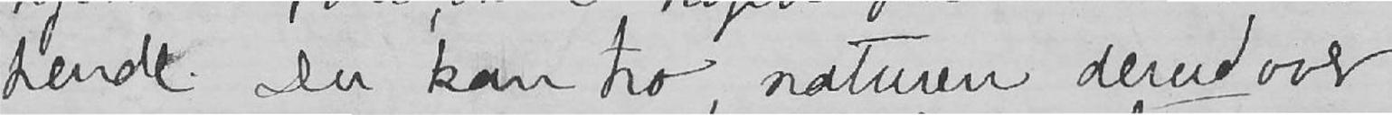hende. Du kan tro, naturen derudover
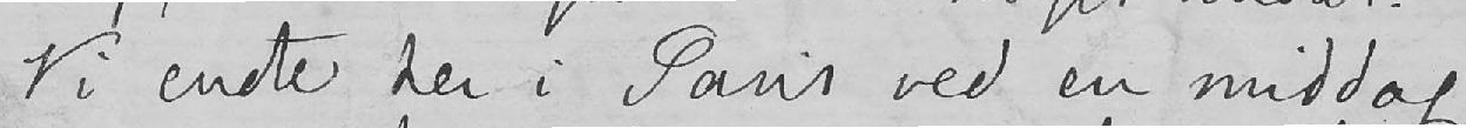Vi endte her i Paris ved en middag
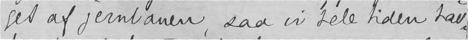get af jernbanen, saa vi hele tiden hav-
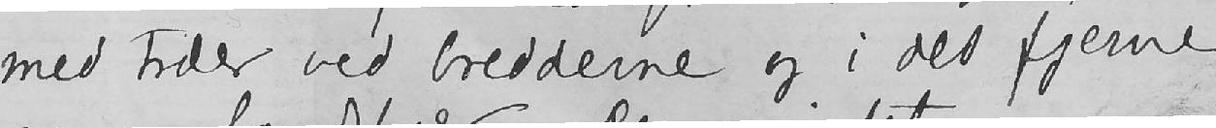med træer ved bredderne og i det fjerne
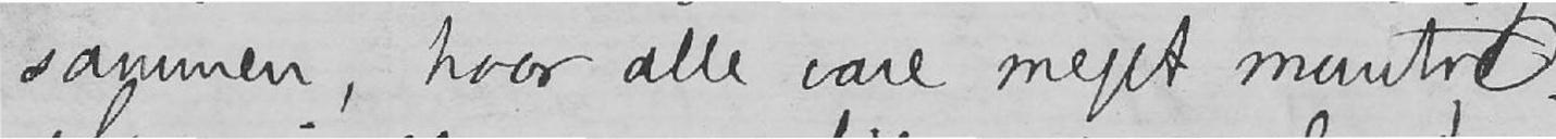sammen, hvor alle vare meget muntre.
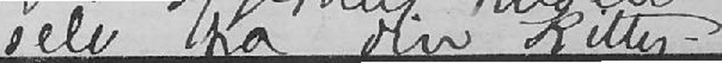selv fra din Kitty.
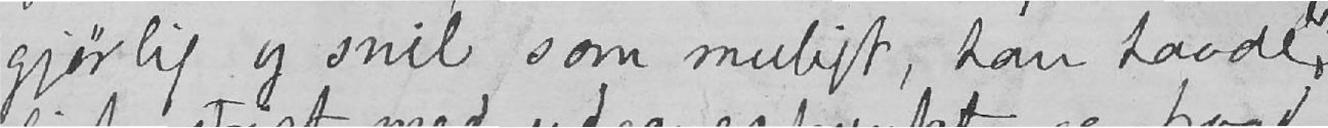gjørlig og snil som muligt, han havde
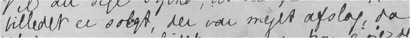billedet er solgt, der var meget afslag, da
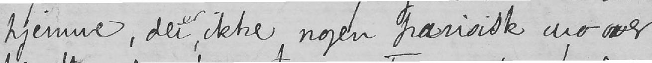hjemme, der er ikke nogen parisisk uro over
